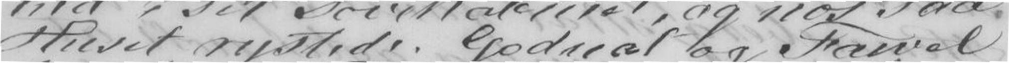Huset rystede. Godnat og Farvel
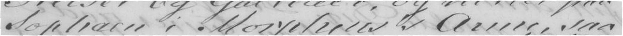Sophaen i Morphens's Arme, saa
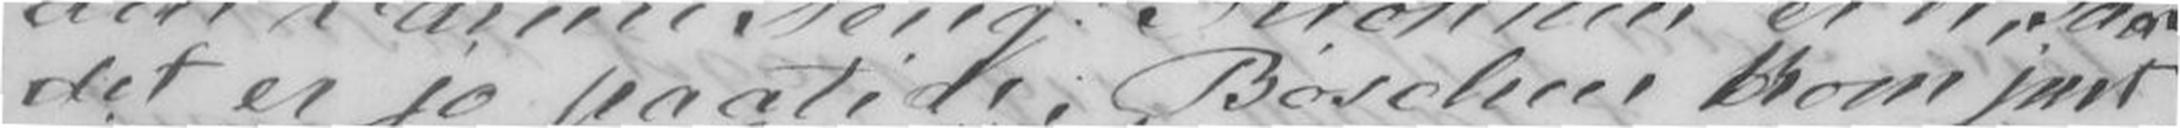det er jo paatide; Bøschen kom just
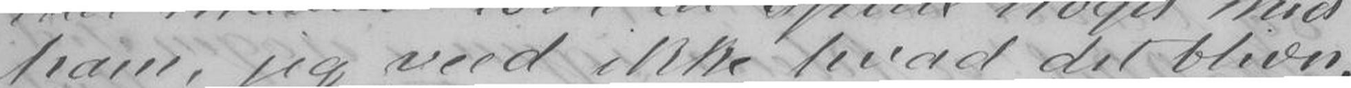ham, jeg veed ikke hvad det bliver,
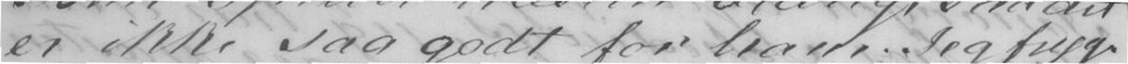er ikke saa godt for ham. Jeg fryg-
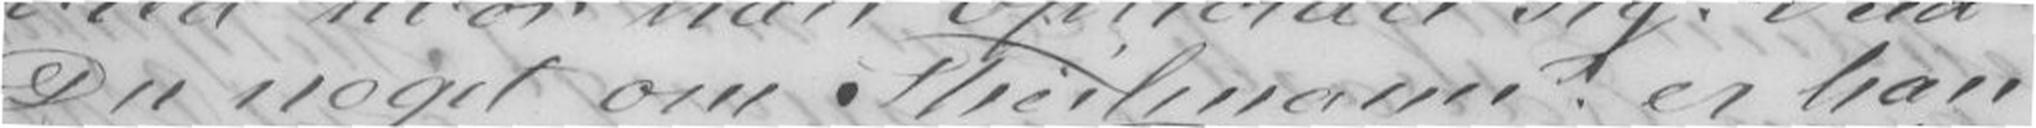Du noget om Theilmann? er han
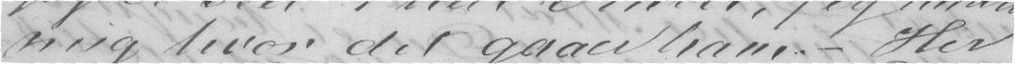mig hvor det gaaer ham. - Her
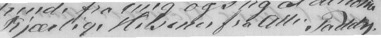kjærlige Hilsener fra Alle. Paddy.
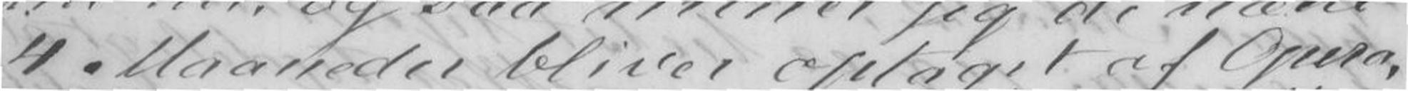4 Maaneder bliver optaget af Opera,
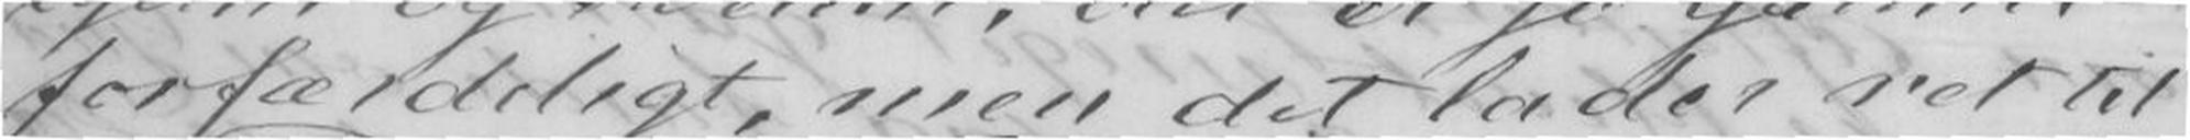forfærdeligt, men det lader ret til
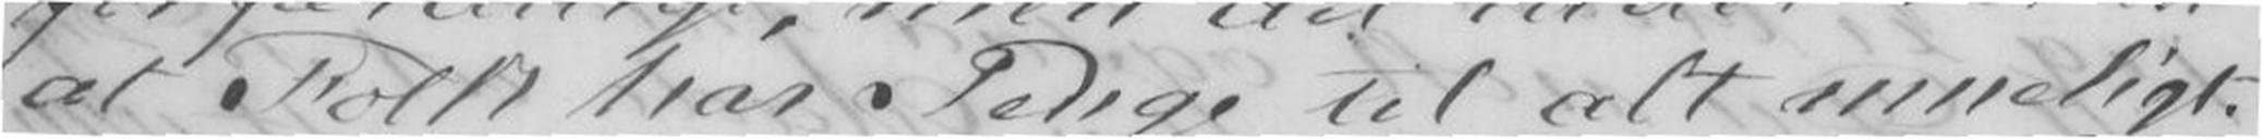at Folk har Penge til alt mueligt.
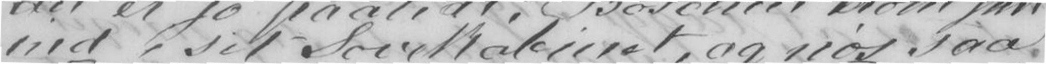ind i sit Sovekabinet, og nøs saa
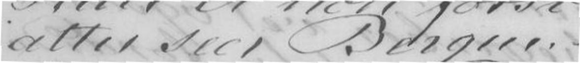atter seer Bergen.
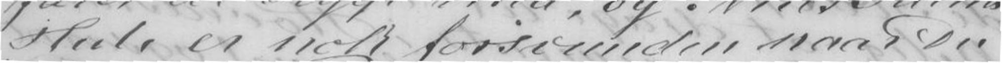Hule er nok forsvunden naar Du
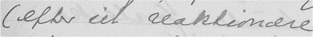(efter sit reaktionære
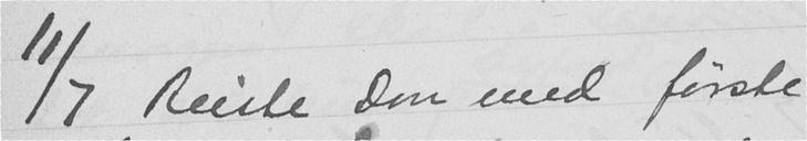11/7 Reiste Don med første
